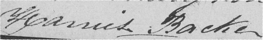Harriet Backer
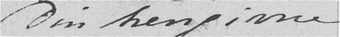Din hengivne
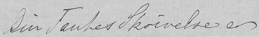Din Tantes Skrivelse er
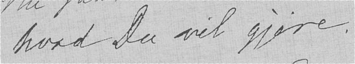hvad Du vil gjøre.
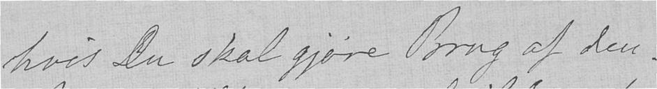hvis Du skal gjøre Brug af den.
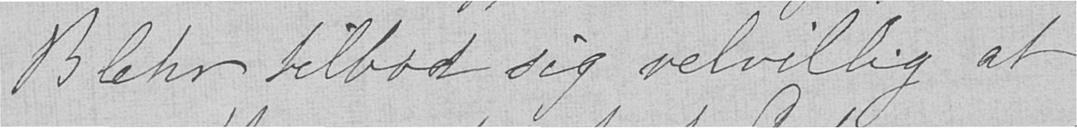Blehr tilbød sig velvillig at
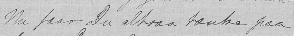Nu faar Du altsaa tænke paa
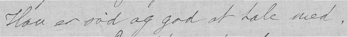Han er sød og god at tale med.
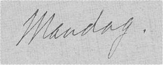Mandag.
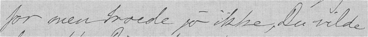for men troede jo ikke, Du vilde
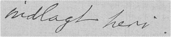vedlagt heri.
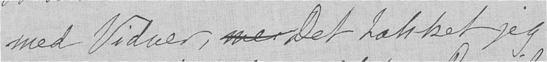med Vidner,  det takket jeg
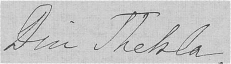Din Thekla.
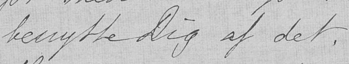benytte Dig af det.
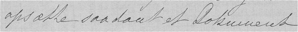opsætte saadant et Dokument
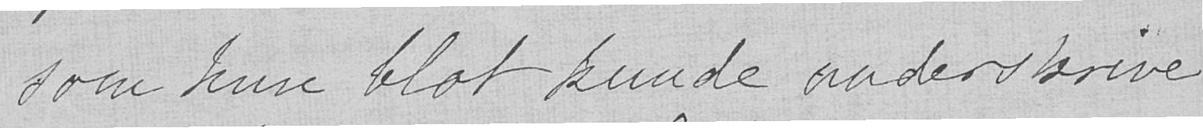som hun blot kunde underskrive
